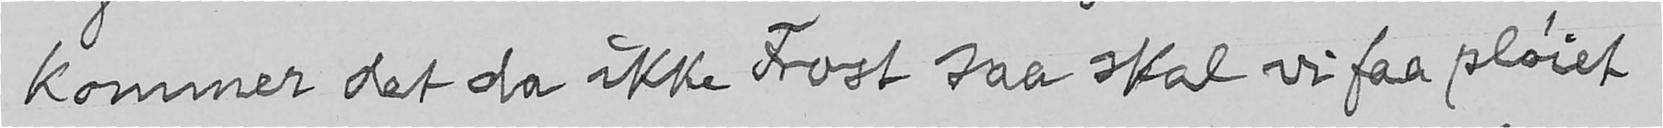kommer det da ikke Frost saa skal vi faa pløiet
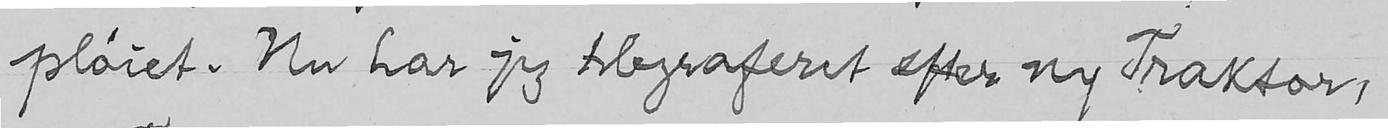pløiet. Nu har jeg telegraferet efter ny Traktor,
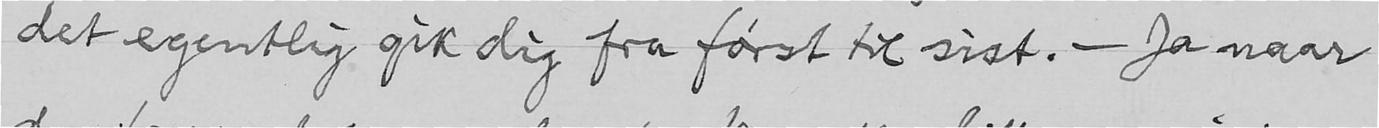det egentlig gik dig fra først til sist. - Ja naar
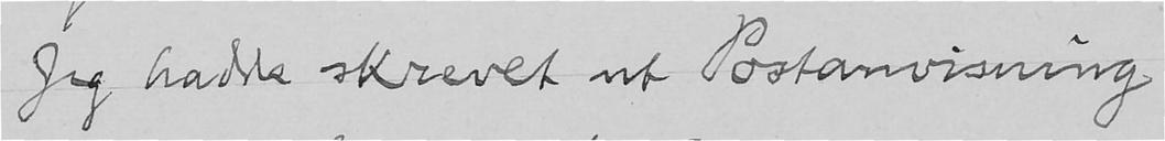Jeg hadde skrevet ut Postanvisning
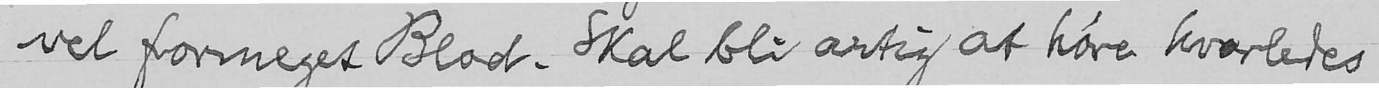vel formeget Blod. Skal bli artig at høre hvorledes
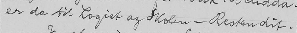er da til Logiet og Skolen - Resten dit.
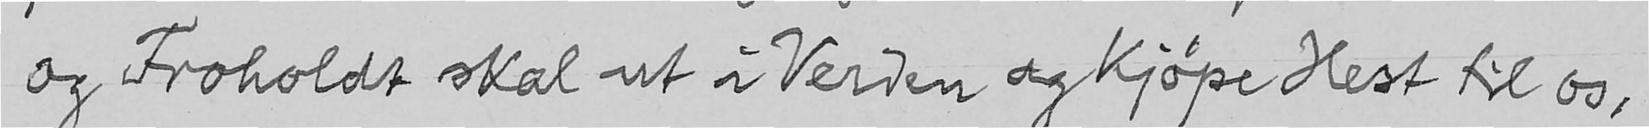og Froholdt skal ut i Verden og kjøpe Hest til os,
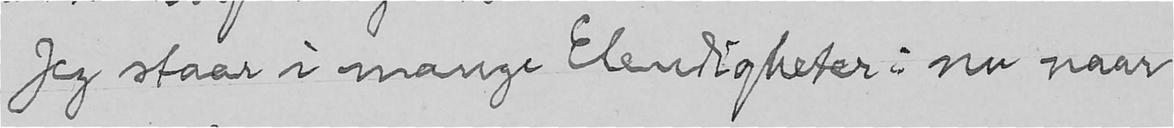Jeg staar i mange Elendigheter: nu naar
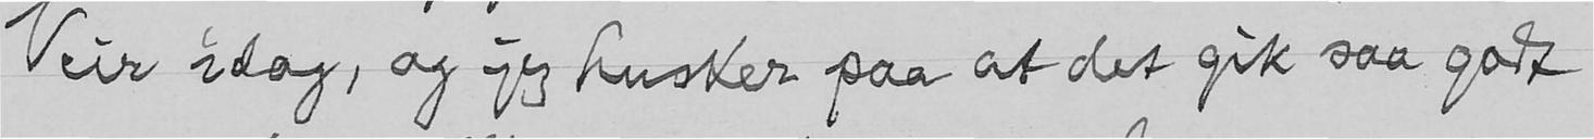Veir idag, og jeg husker paa at det gik saa godt
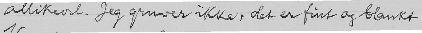allikevel. Jeg gruver ikke, det er fint og blankt
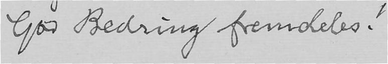God Bedring fremdeles!
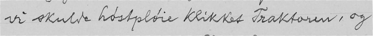vi skulde høstpløie klikket Traktoren, og
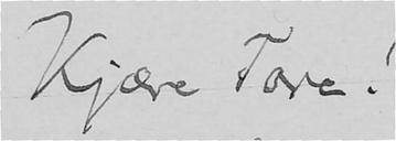Kjære Tore!
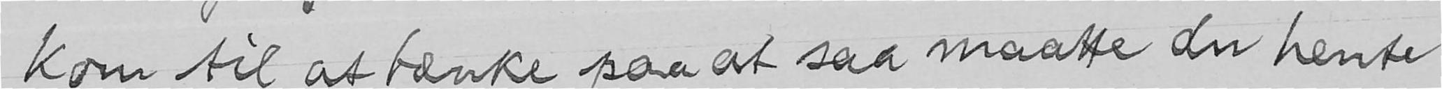kom til at tænke paa at saa maatte du hente
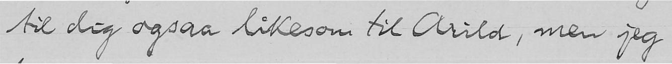til dig ogsaa likesom til Arild, men jeg
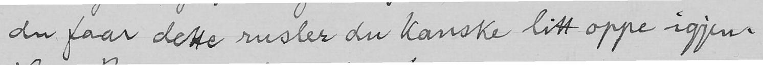du faar dette rusler du kanske litt oppe igjen.
